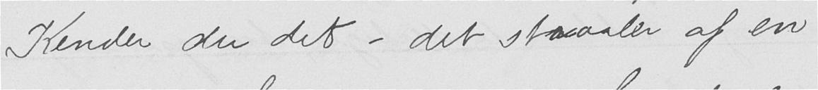Kender du det - det straaler af en
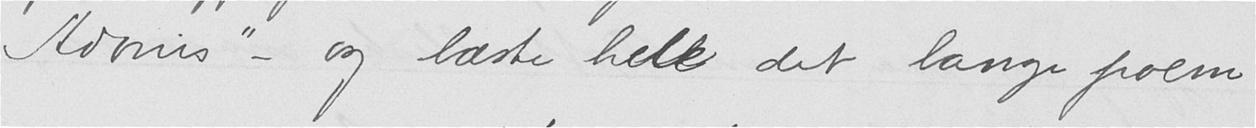Adonis" - og læste hele det lange poem
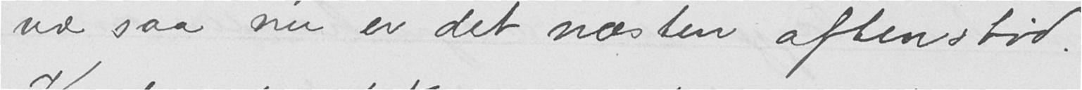ud saa nu er det næsten aftenstid.
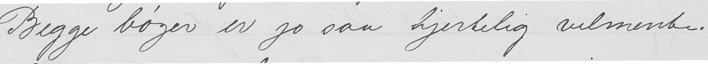Begge bøger er jo saa hjertelig velmente.
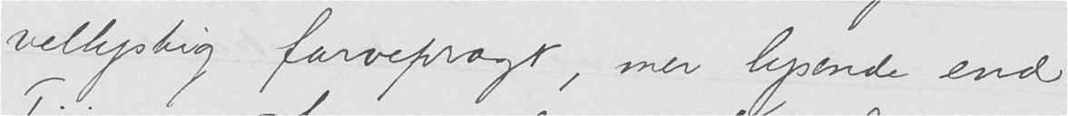vellystig farvepragt, mer lysende end
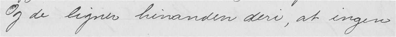Og de ligner hinanden deri, at ingen
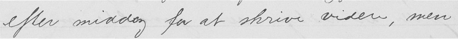efter middag for at skrive videre, men
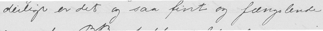deiligt er det og saa fint og fængslende
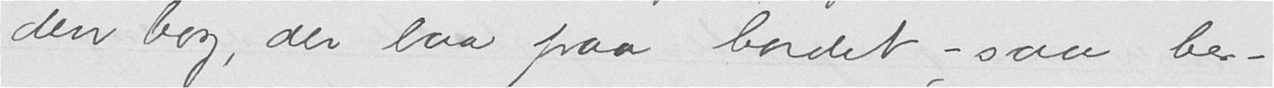den bog, der laa paa bordet - saa be-
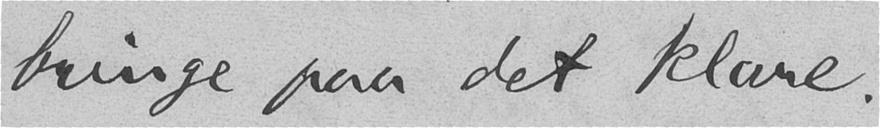bringe paa det klare.
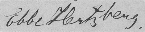Ebbe Hertzberg.
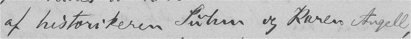af historikeren Suhm og Karen Angell,
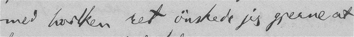med hvilken ret ønskede jeg gjerne at
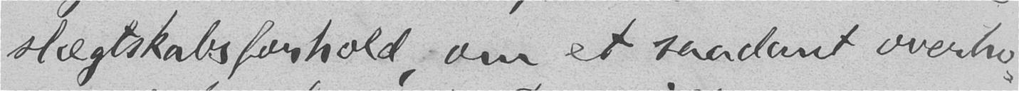slægtskabsforhold, om et saadant overho-
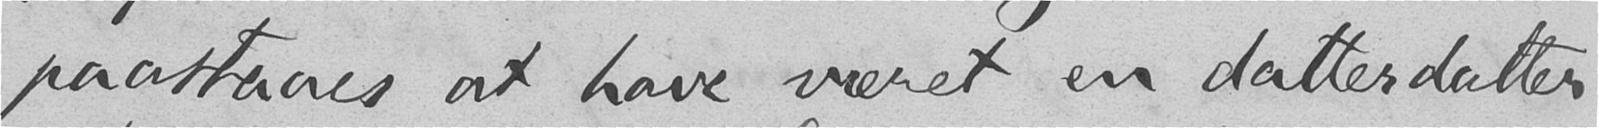paastaaes at have været en datterdatter
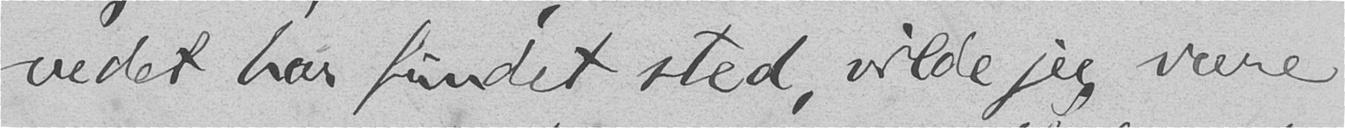vedet har fundet sted, vilde jeg være
